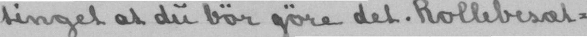tinget at du bør gøre det. Rollebesæt-
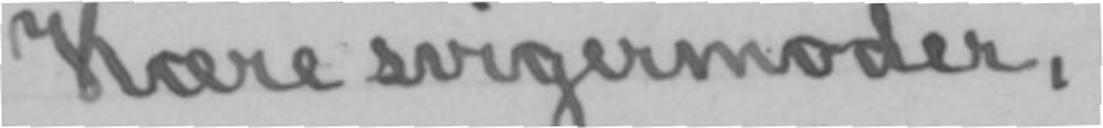Kære svigermoder,
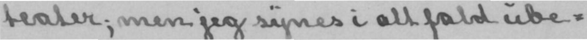teater; men jeg synes i alt fald ube-
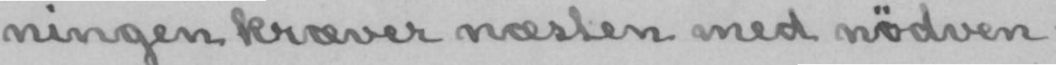ningen kræver næsten med nødven-
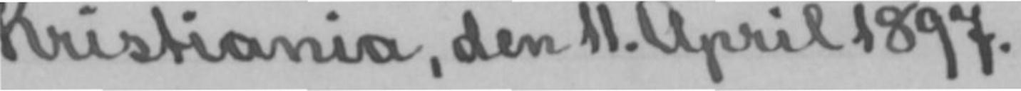Kristiania, den 11. April 1897.
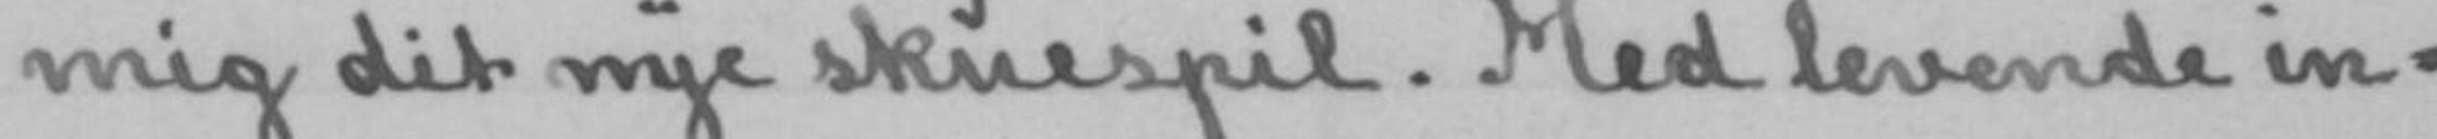mig dit nye skuespil. Med levende in-
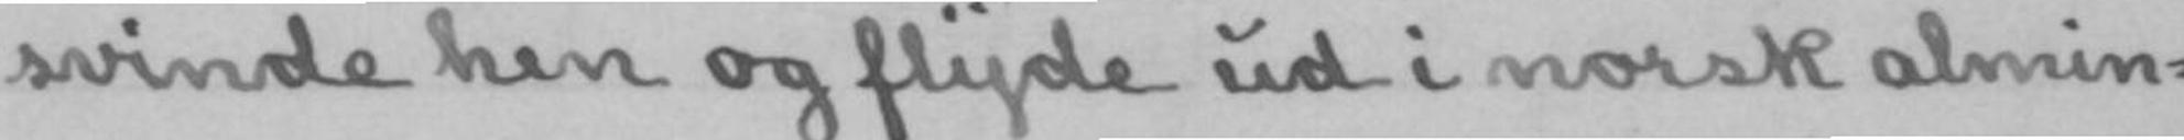svinde hen og flyde ud i norsk almin-
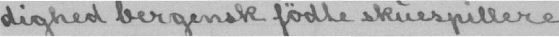dighed bergensk fødte skuespillere
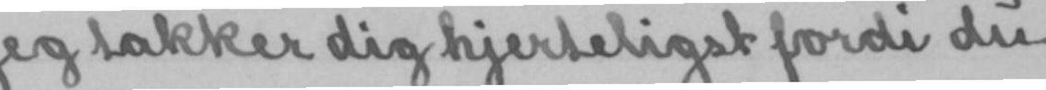Jeg takker dig hjerteligst fordi du
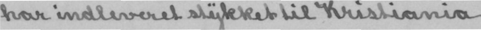har indleveret stykket til Kristiania
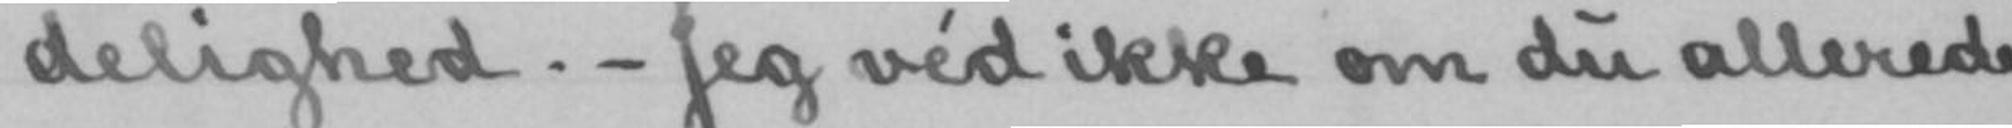delighed.- Jeg véd ikke om du allerede
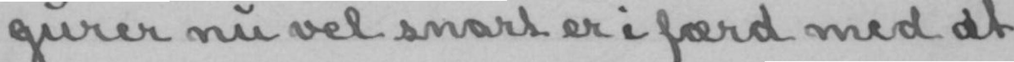gurer nu vel snart er i færd med at
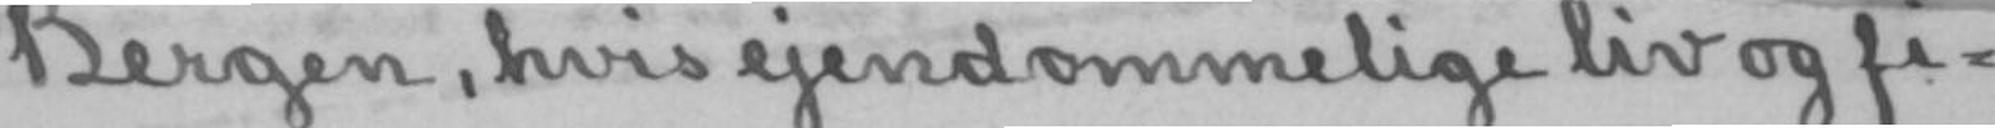Bergen, hvis ejendommelige liv og fi-
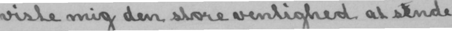viste mig den store venlighed at sende
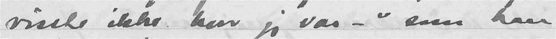visste ikke hvor jeg var -" som han
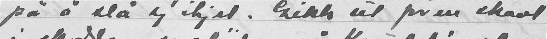på i slå sig ihjel. Gikk ut for en skrent
